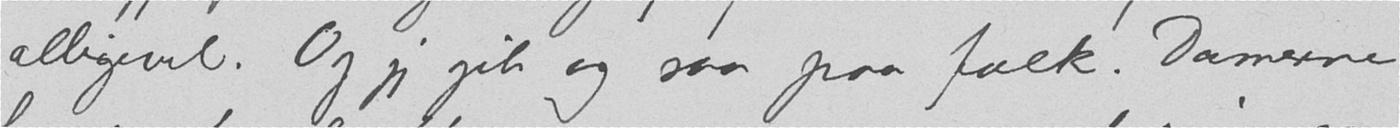alligevel. Og jeg gik og saa paa folk. Damerne
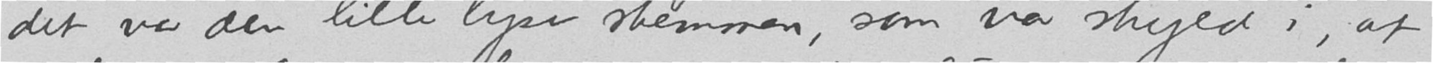det var den lille lyse stemmen, som var skyld i, at
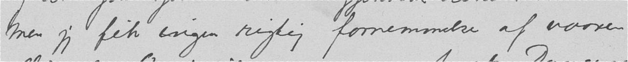Men jeg fik ingen rigtig fornemmelse af vaaren
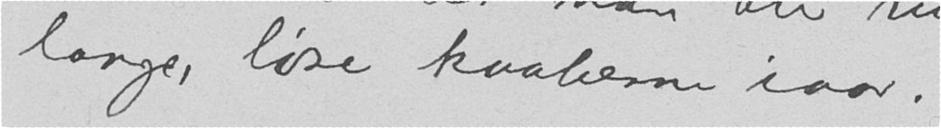lange, løse kaaberne iaar.
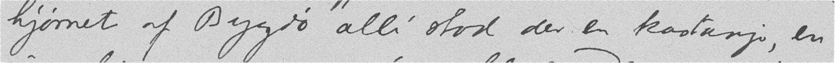hjørnet af Bygdø alle stod der en kastanje, en
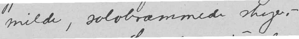milde, sølvbræmmede skyer. -
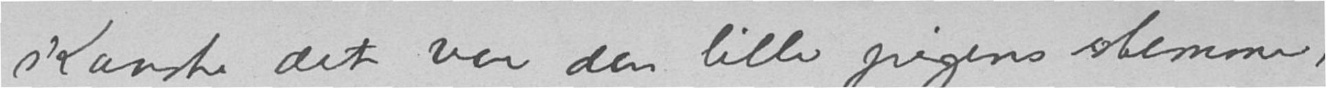Kanske det var den lille pigens stemme,
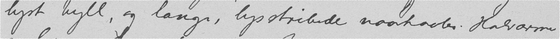lyst tyll og lange, lysstribede vaarkaaber. Halværmer
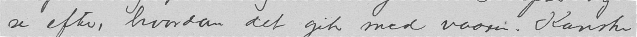se efter, hvordan det gik med vaaren. Kanske
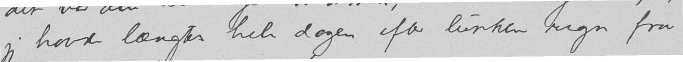jeg havde længtes hele dagen efter lunken regn fra
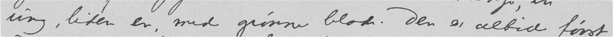ung, liden en, med grønne blade. Den er altid først
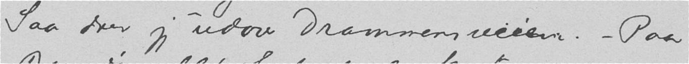Saa drev jeg udover Drammensveien. - Paa
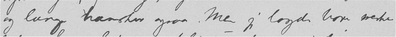og lange hansker ogsaa. Men jeg lagde bare merke
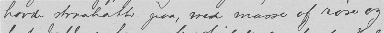havde straahatter paa, med masser af roser og
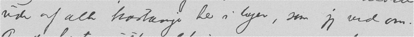ude af alle kastanjer her i byer, som jeg ved om.
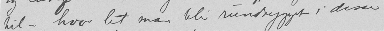til - hvor let man blir rundrygget i disse
